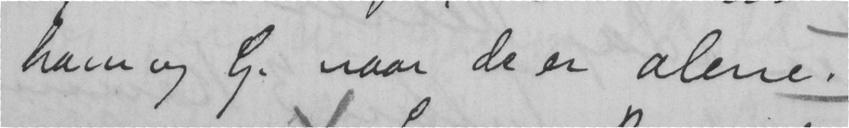ham og G. naar de er alene.
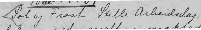Sol og Frost. Stille arbeidsdag.
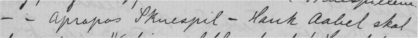- - Apropos Skuespil - Hank Aabel skal
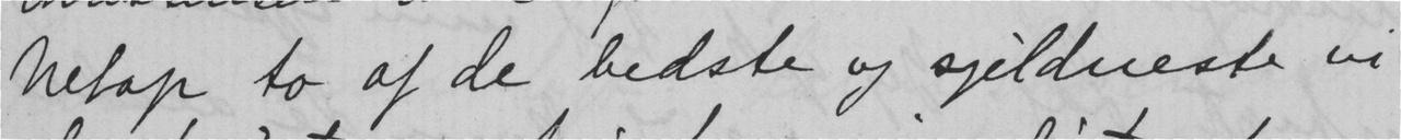Netop to af de bedste og sjeldneste vi
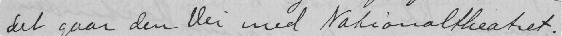det gaar den vei med Nationaltheatret.
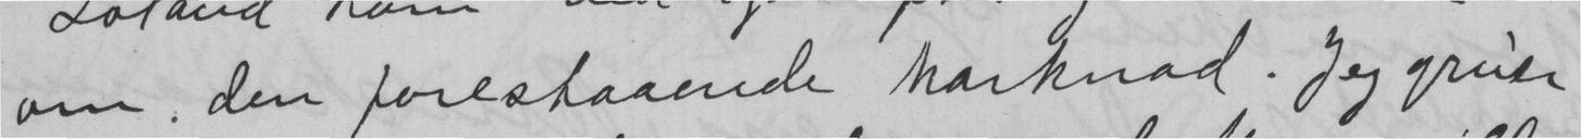om den forestaaende Marknad. Jeg gruer
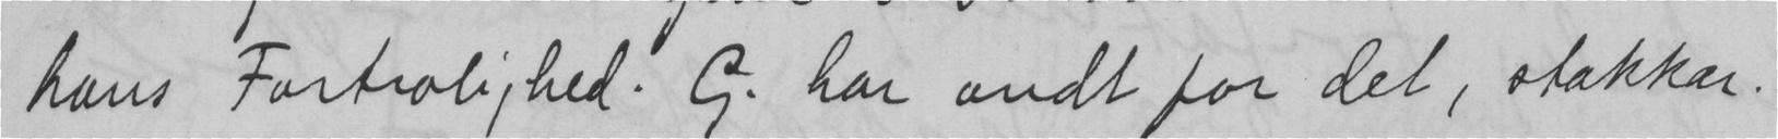hans Fortrolighed. G. har ondt for det, stakkar.
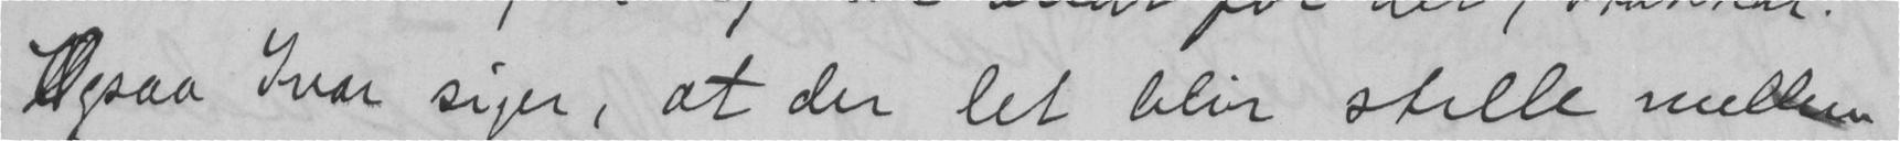Ogsaa Ivar siger, at der let blir stille mellem
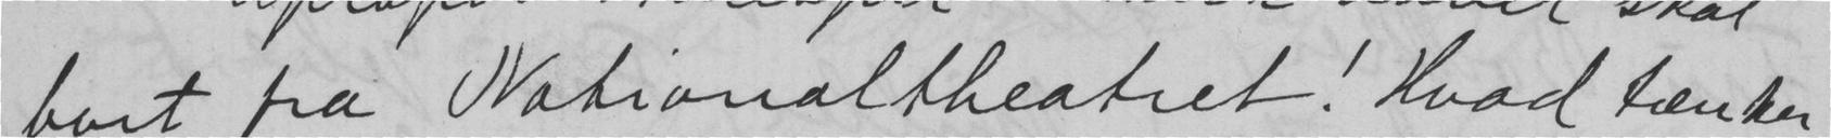bort fra Nationaltheatret! Hvad tænker
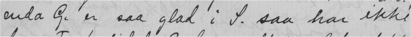enda G. er saa glad i S. saa har ikke
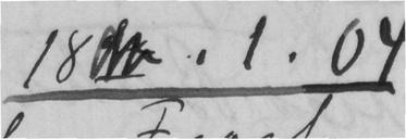18  .1.04
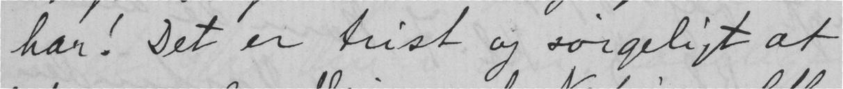har! Det er trist og sørgeligt at
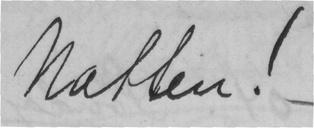Natten!
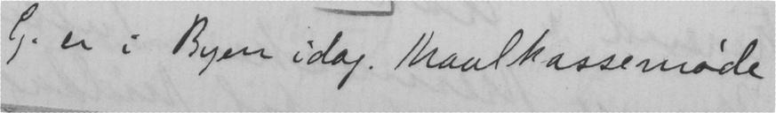G. er i Byen idag. Maalkassemøde
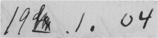19 .1.04
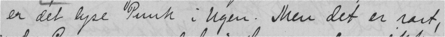er det lyse Punk i Ugen. Men det er rart,
